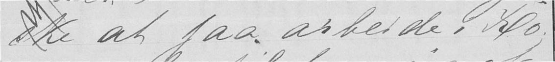ske at faa arbeide i Ro,
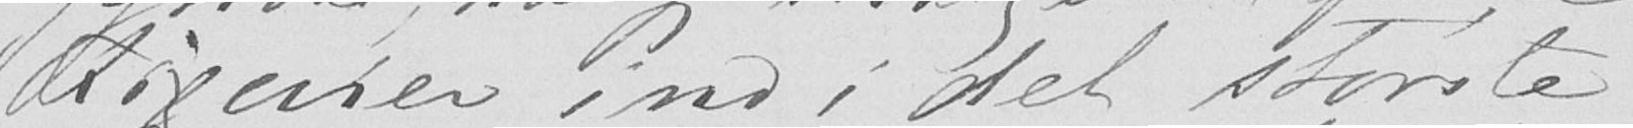Figurer ind i det største
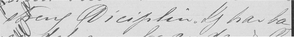streng Disiplin. Jeg har ba-
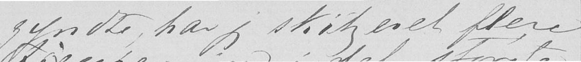gyndte har jeg skitzeret flere
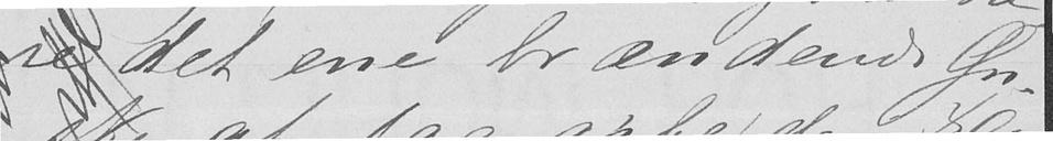re det ene brændende Øn-
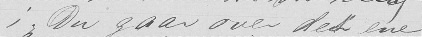i. Du gaar over det ene
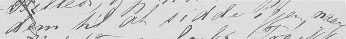dem til at sidde igjen, naar
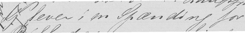Jeg lever i en Spænding for
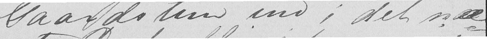Gaardstun ind i det næ-
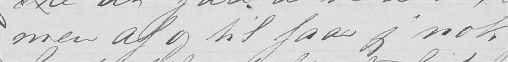men af og til faar jeg nok
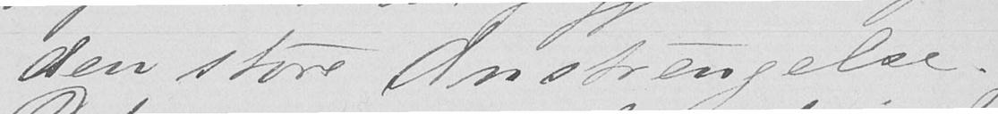den store Anstrengelse.
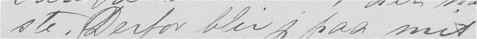ste. Derfor blir jeg paa mit
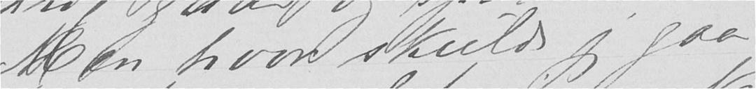Men hvor skulde jeg gaa
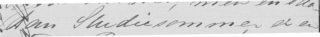dan Studiesommer er en
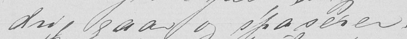drig gaar og spaserer.
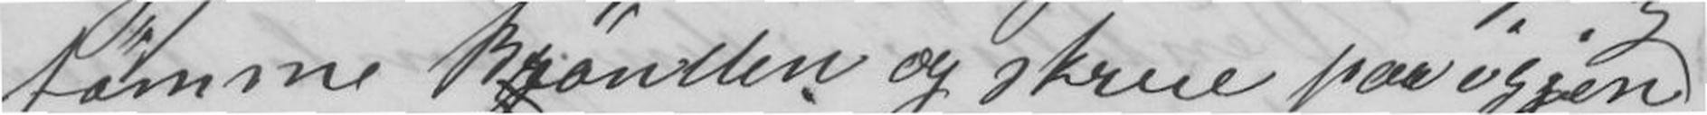tømme Brønden og skrue paa igjen.
